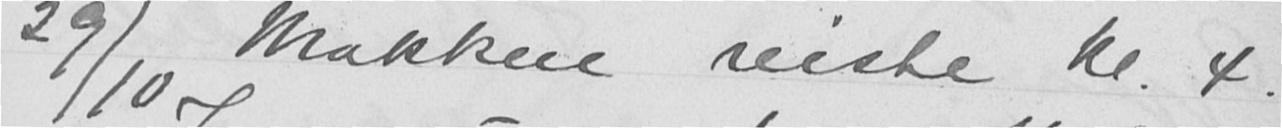29/10 Makken reiste kl. 4.
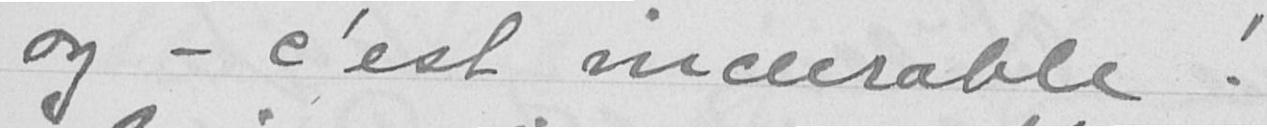og - c'est incurable!
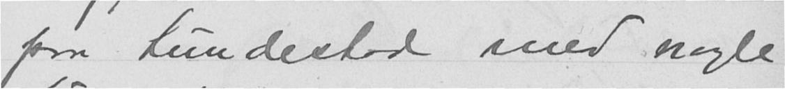paa Lundestad med nogle
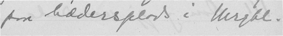paa hædersplads i Mrgbl.
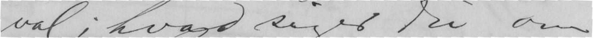val; hvad siger du om
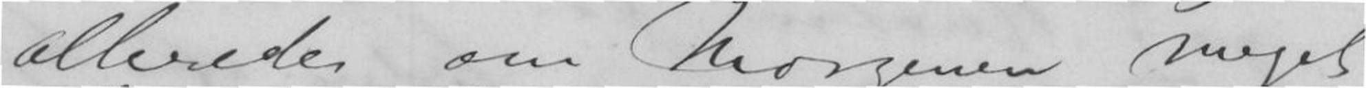allerede om Morgenen meget
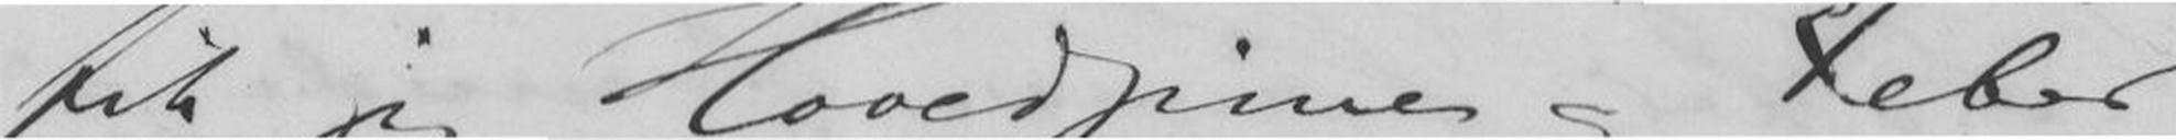fik jeg Hovedpine og Feber
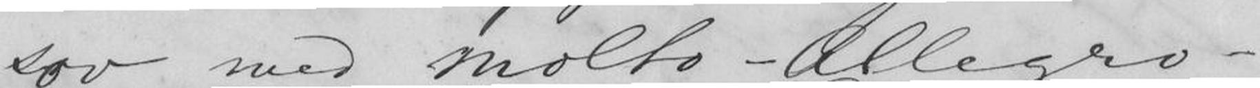sov med Molto-Allegro-
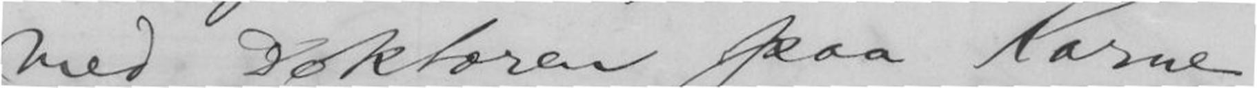med Doktoren paa Karne
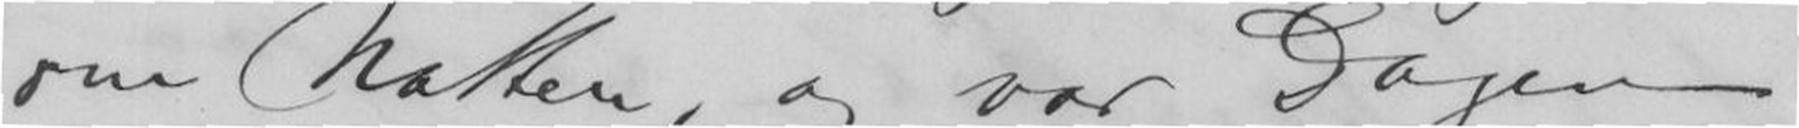om Natten, og var Dagen
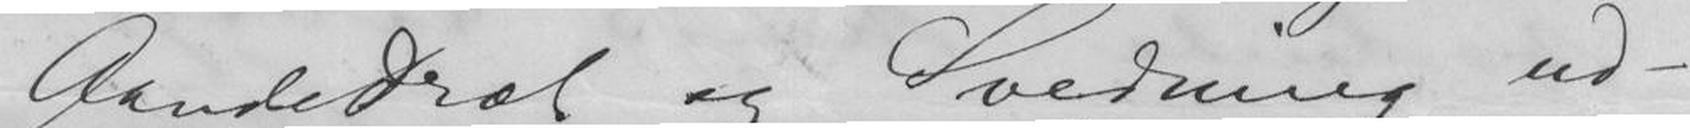Aandedræt og Svedning ud-
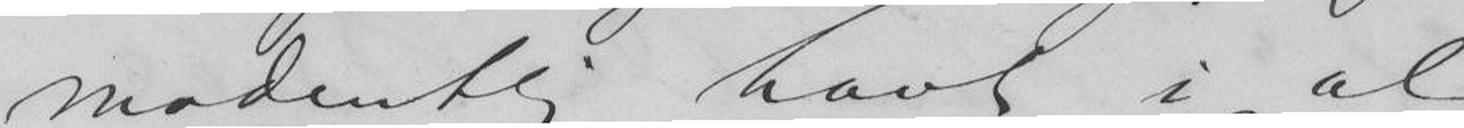modentlig havt i al
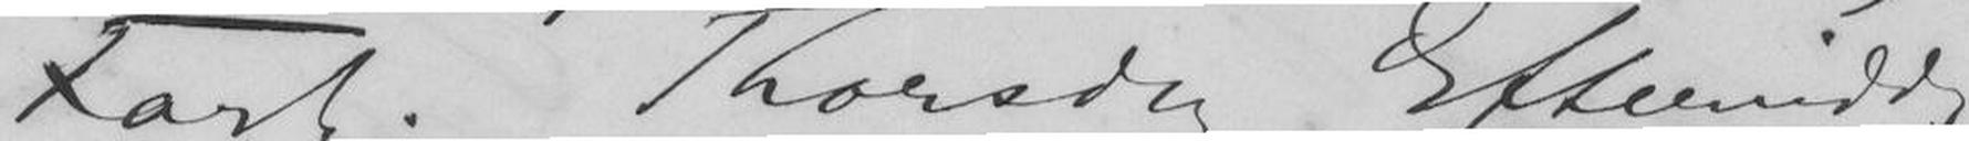Fart. Thorsdag Eftermiddag
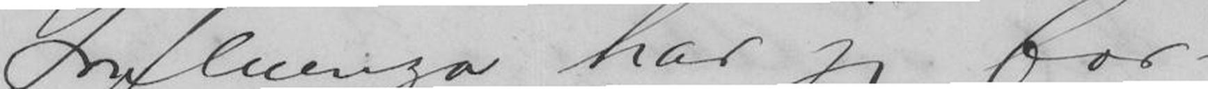Influenza har jeg for-
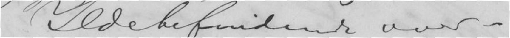og Ildebefinde over-
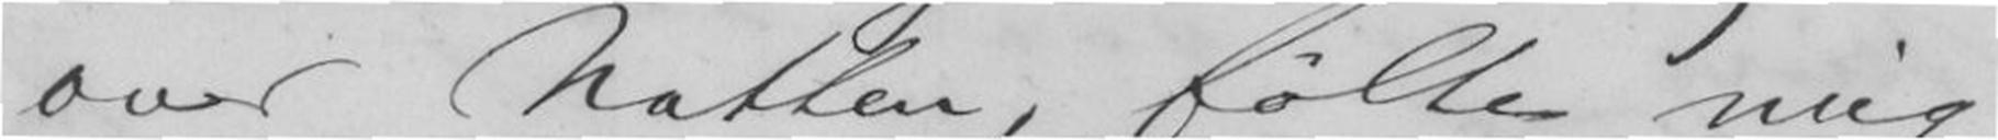over Natten, følte mig
